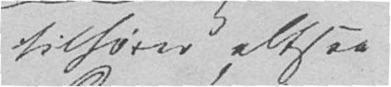tilhører altsaa
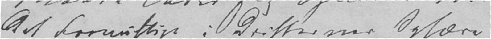det Fornuftige i Drifternes Sphære
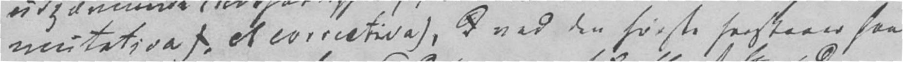mutativa), et corrective), d ved den første forstaaer han
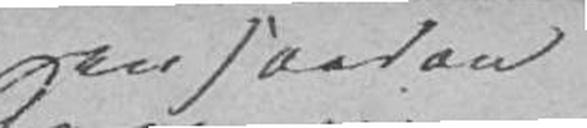en saadan
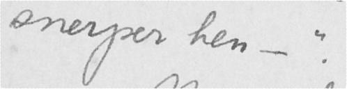snerper hen - ".
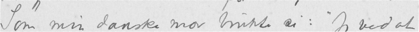Som min danske mor brukte si: "Jeg ved at
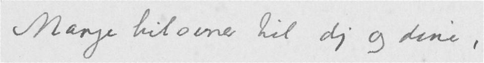Mange hilsener til dig og dine,
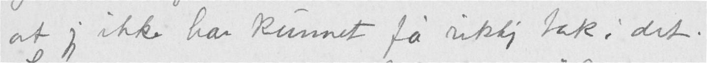at jeg ikke har kunnet få riktig tak i det.
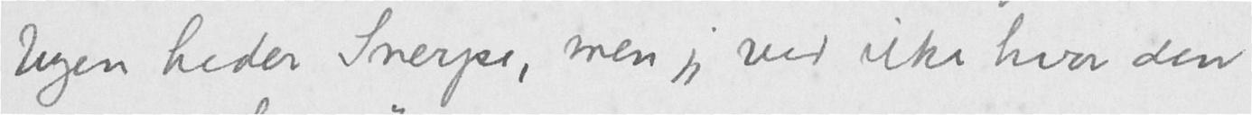byen heder Snerpe, men jeg ved ikke hvor den
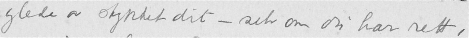glede av stykket dit - selv om du har rett i
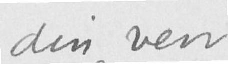din ven
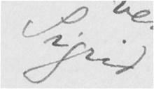Sigrid
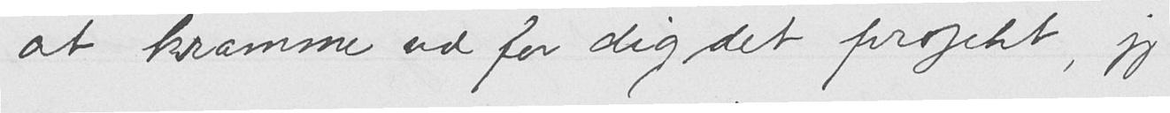at kramme ud for dig det projekt, jeg
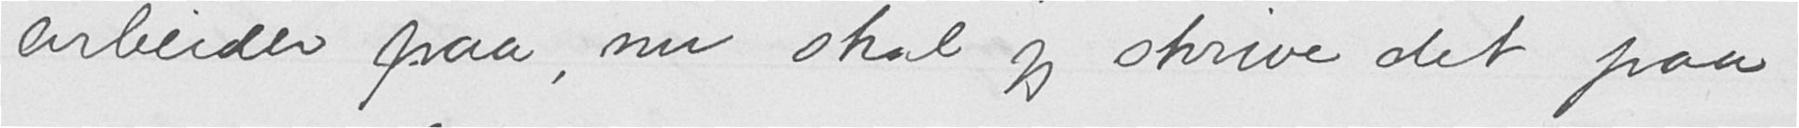arbeider paa, nu skal jeg skrive det paa
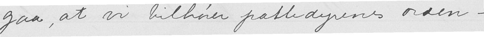gaa, at vi tilhører pattedyrenes orden -
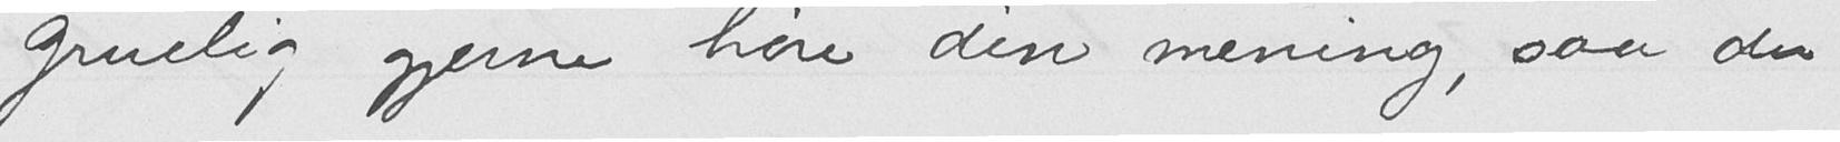gruelig gjerne høre din mening, saa du
